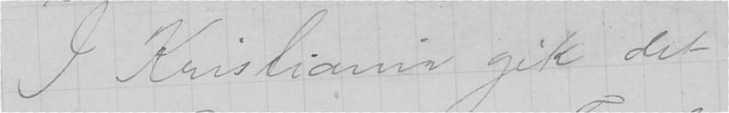I Kristiania gik det
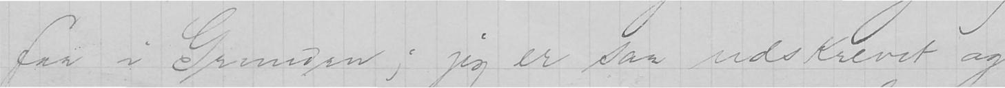faa i Grunden; jeg er saa udskrevet og
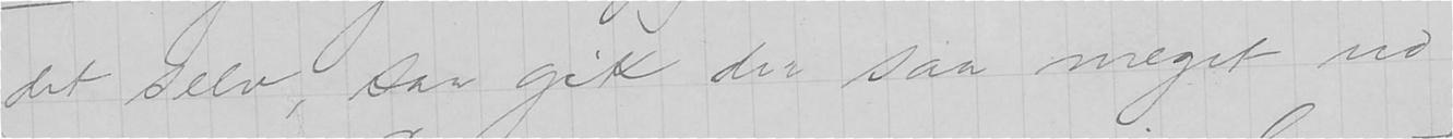det selv, saa gik der saa meget ud
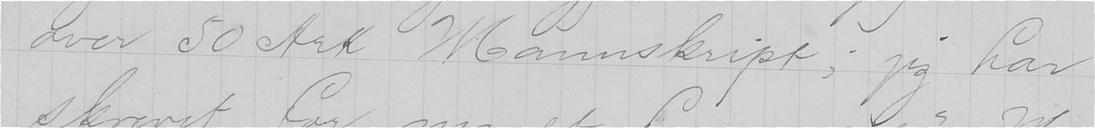over 50 Ark Manuskript; jeg har
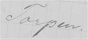Torpen.
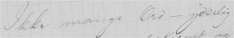Ikke mange Ord - yderlig
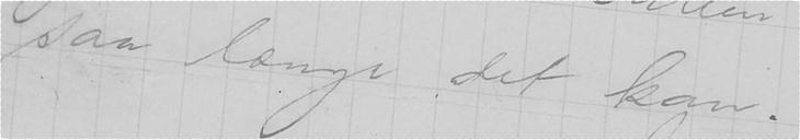saa længe det kan.
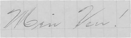Min Ven!
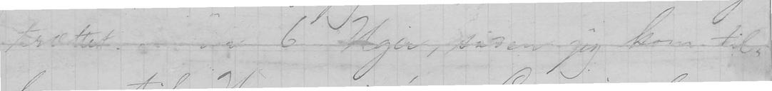trættet. Paa 6 Uger, siden jeg kom til-
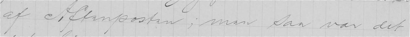af Aftenposten; men saa var det
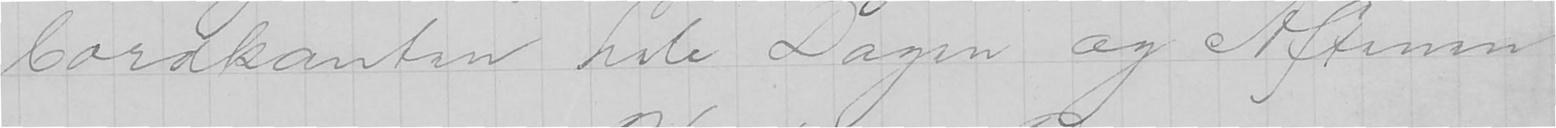bordkanten hele Dagen og Aftenen
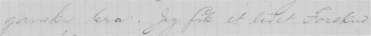ganske bra. Jeg fik et lidet Forskud
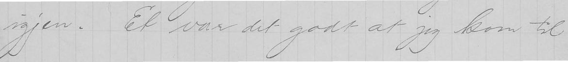igjen. Et var det godt at jeg kom til
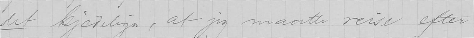det kjedelige, at jeg maatte reise efter
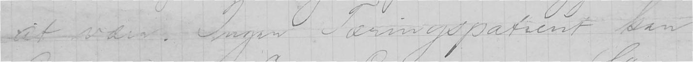at være. Ingen Tæringspatient kan
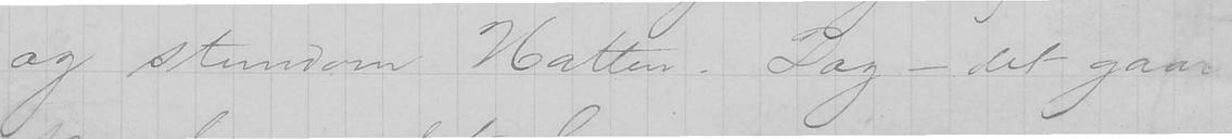og stundom Natten. Dog - det gaar
